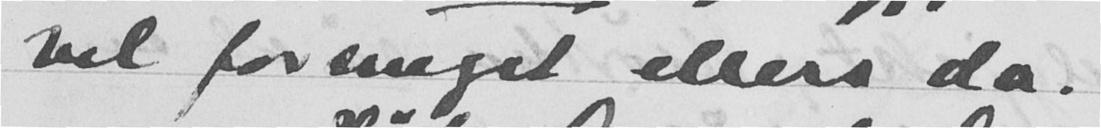vel formeget ellers da.
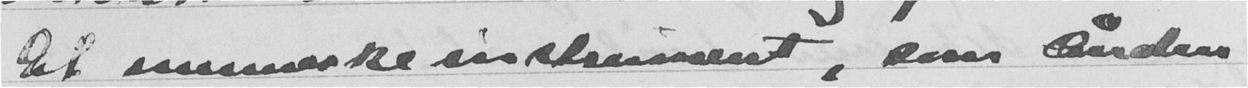Det menneskeinstrument, som Ånden
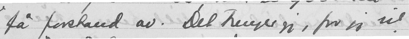få forstand av. Det trenger jeg, for jeg vil
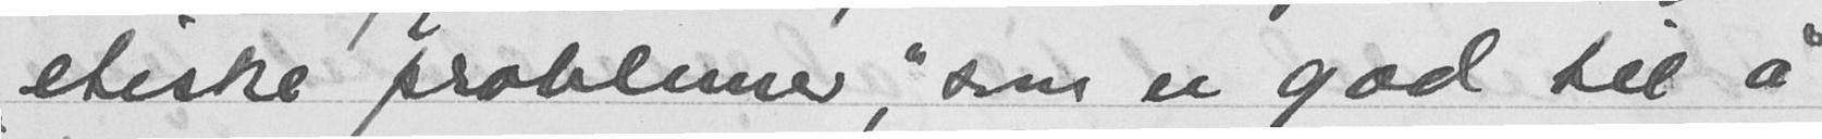"etiske problemer" som er god til i
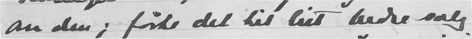om den; førte det til litt bedre salg
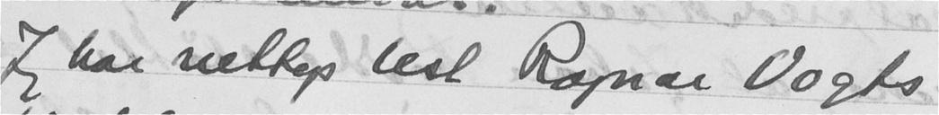Jeg har nettop lest Ragnar Vogts
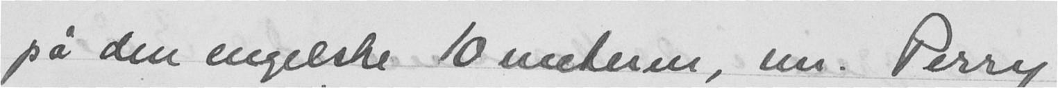på den engelske 10 meteren, sen. Perry
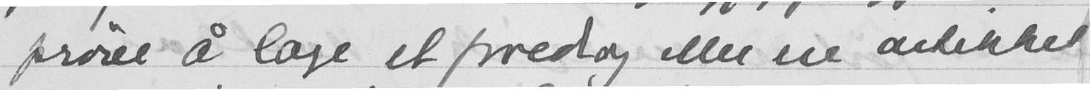prøve å lage et foredrag eller en artikkel
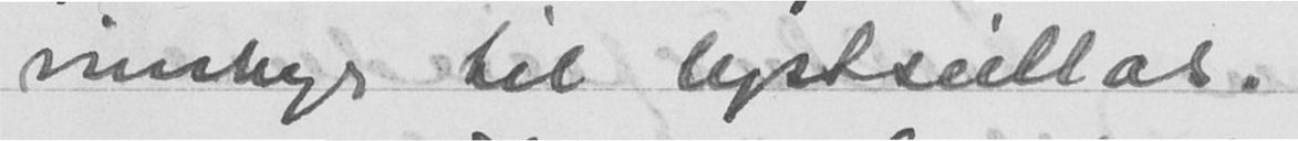innbyr til lystseillas.
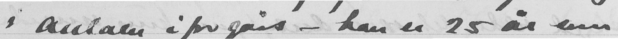i Aulaen iforgårs - han er 25 år som
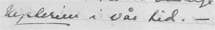keplerine i vår tid. -
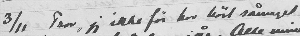3/11 Tror, jeg ikke før har hørt såmeget
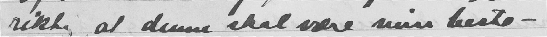riktig at denne skal være min beste -
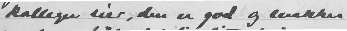kolleger sier, den er god og snakker
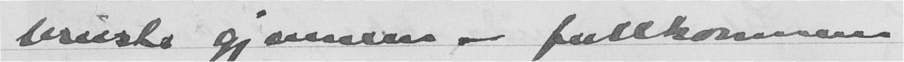bruste gjennom - fullkommen
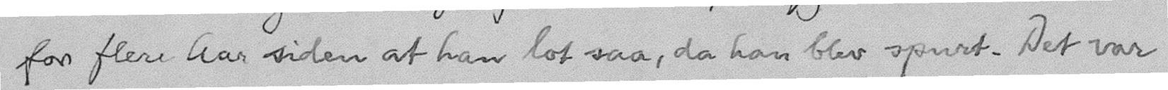for flere Aar siden at han lot saa, da han blev spurt. Det var
